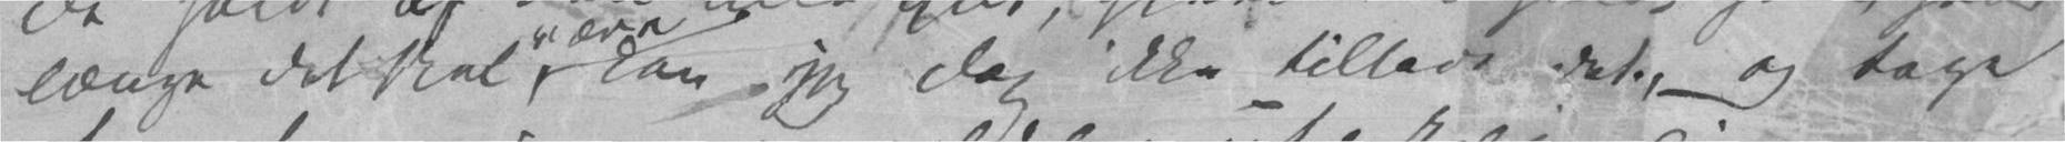længe det skal være, kan jeg dog ikke tillade det, og tage
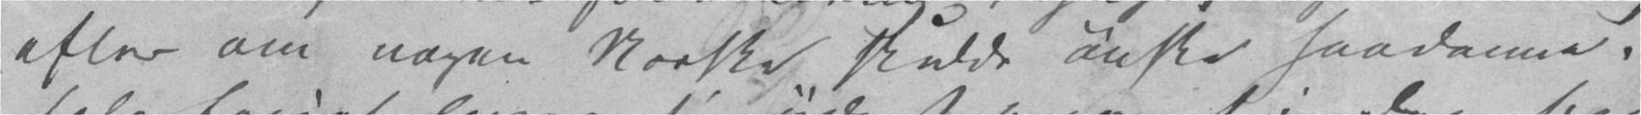efter om nogen Norske skulde ønske saadanne.
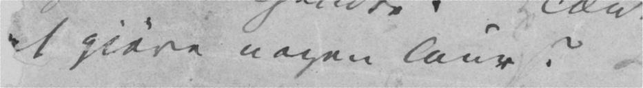at gjøre nogen Tour?
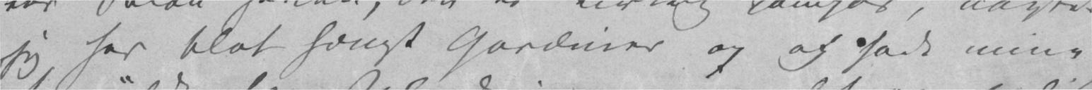jeg har blot hængt Gardiner op og sadt mine
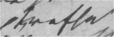treffe
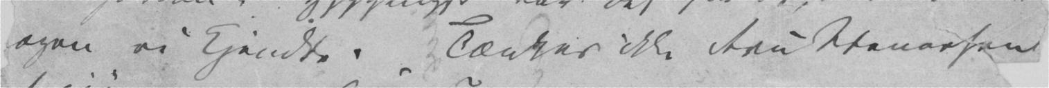ogen vi kjendte. Tænker ikke Fru Stenersen
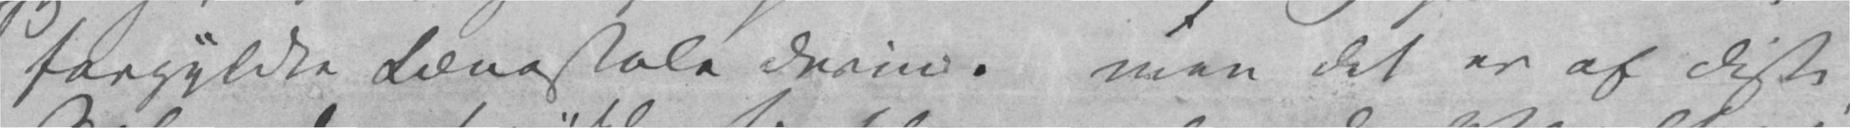forgyldte Lænestole derind. men det er af disse
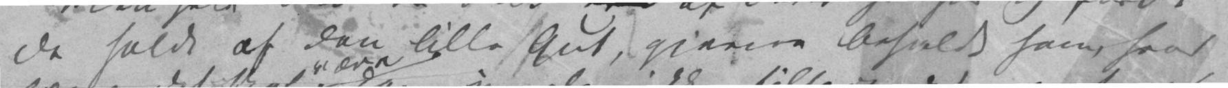de holdt af den lille Gut, gjerne beholdt ham, hvor
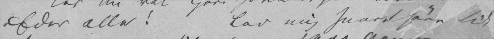Eder alle! Lad mig snart høre lidt
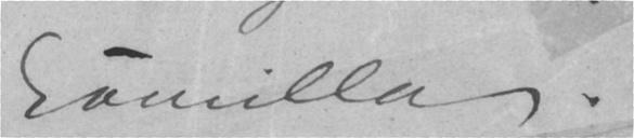Camilla.
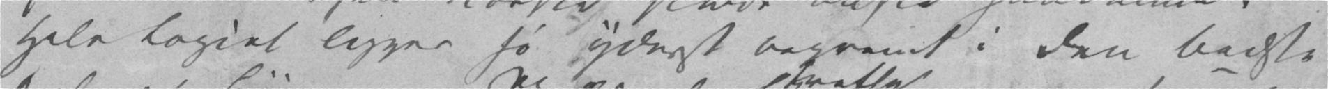Hele Logiet ligger jo yderst beqvemt i den bedste
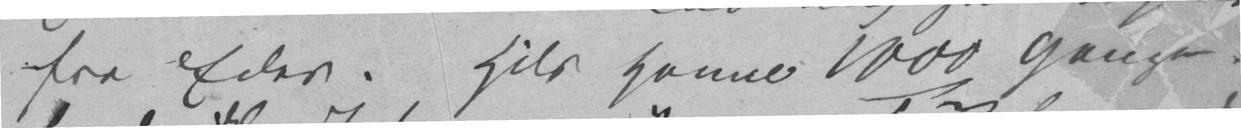fra Eder. Hils Hanne 1000 Gange.
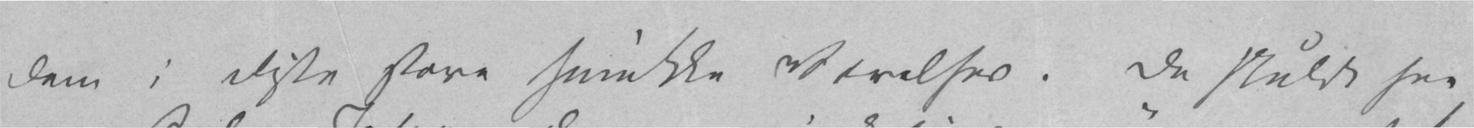dem i disse store smukke Værelser. De skulde see
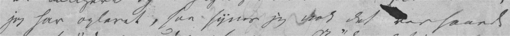jeg har oplevet, saa synes jeg nok det var haardt
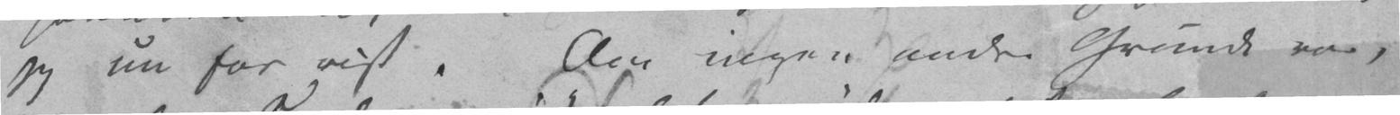jeg nu for vist. Om ingen andre Grunde var,
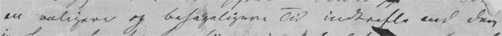en roligere og behageligere Tid indtreffe end den
